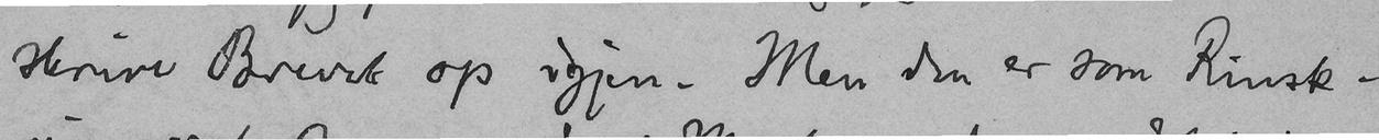skrive Brevet op igjen. Men den er som Rinsk-
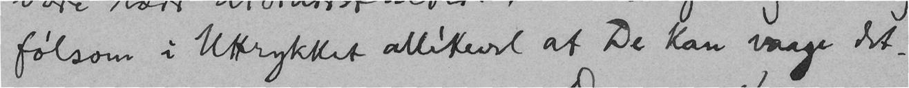følsom i Uttrykket allikevel at De kan vaage det.
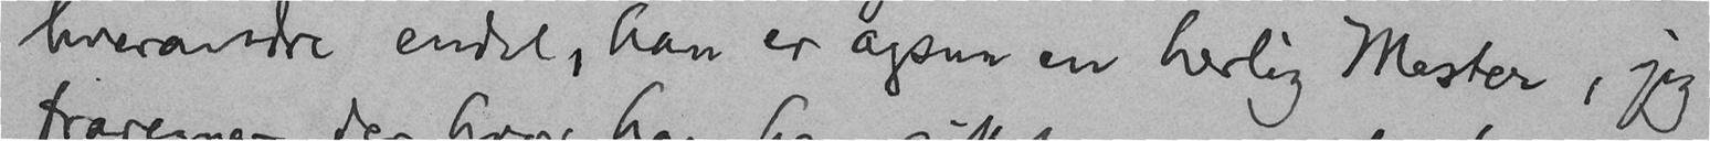hverandre endel, han er ogsaa en herlig Mester, jeg
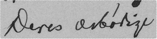Deres ærbødige
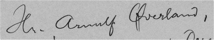Hr. Arnulf Øverland,
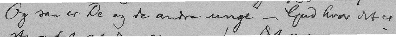Og saa er De og de andre unge - Gud hvor det er
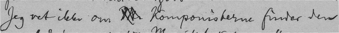Jeg vet ikke om M Komponisterne finder den
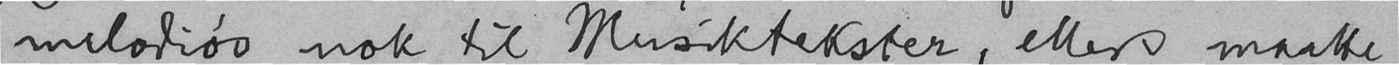melodiøs nok til Musiktekster, ellers maatte
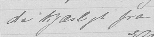de Kjærligt fra
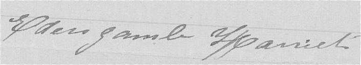Eders gamle Harriet
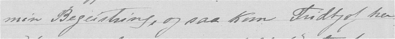min Begeistring, og saa kom Fridtjof her-
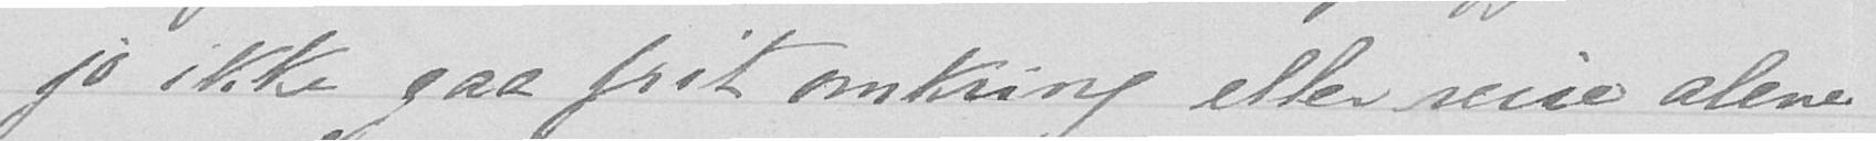jo ikke gaa frit omkring eller reise alene
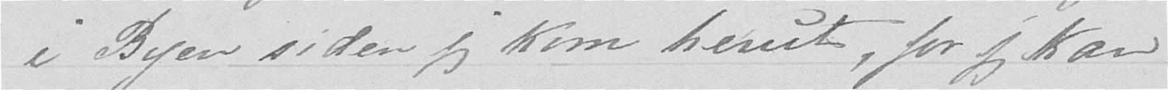i Byen siden jeg kom herut, for jeg kan
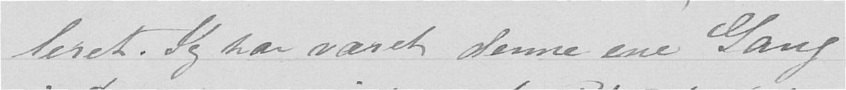leret. Jeg har været denne ene Gang
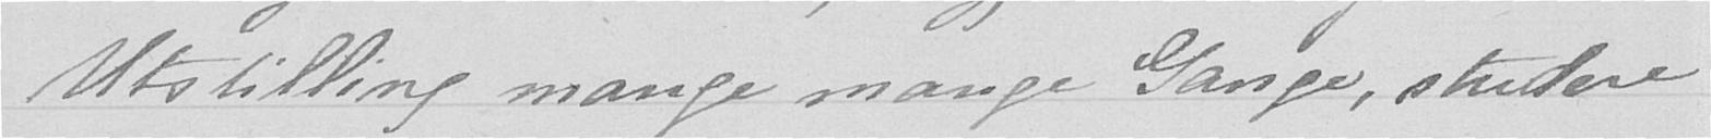Utstilling mange mange Gange, studere
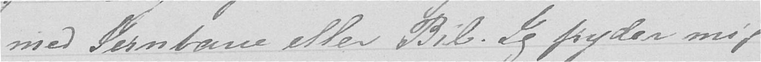med Jernbane eller Bil. Jeg fryder mig
